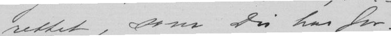rettet, som Du har for-
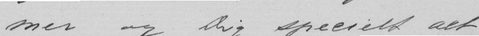mer og Dig specielt alt
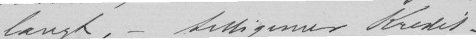langt, - tilligemed Kredit-
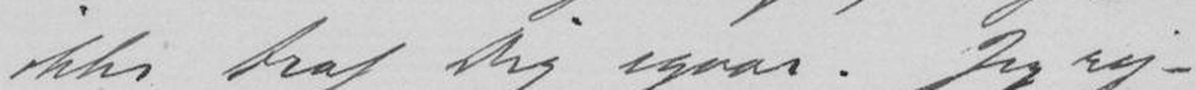ikke traf Dig igaar. Jeg rej-
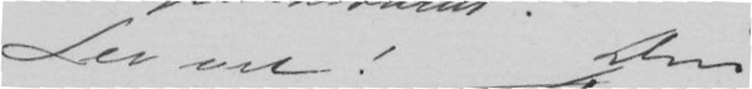Lev vel Din
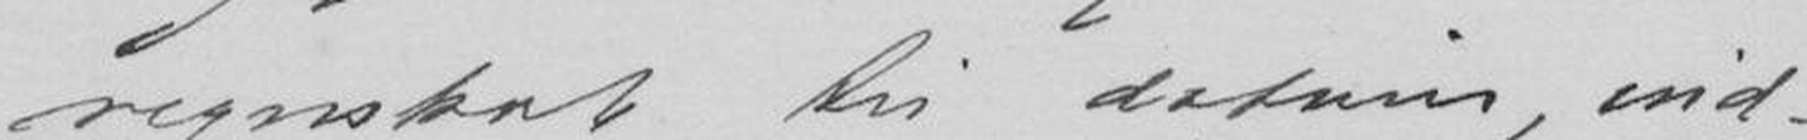regnskab til datum, ind-
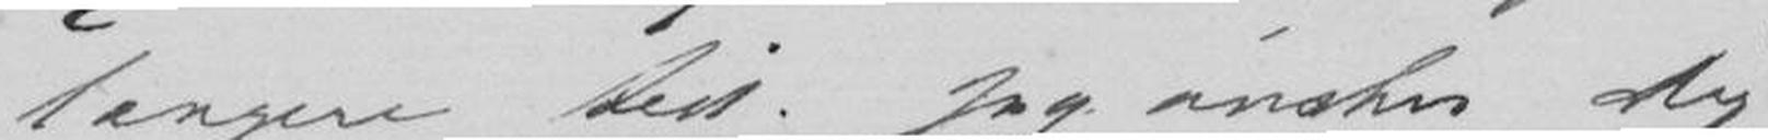længere tid. Jeg ønsker Dig
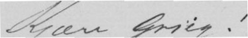Kjære Grieg!
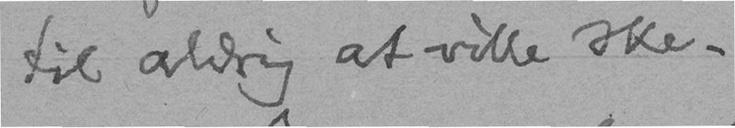til aldrig at ville ske.
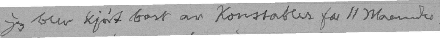jeg blev kjørt bort av Konstabler for 11 Maaneder
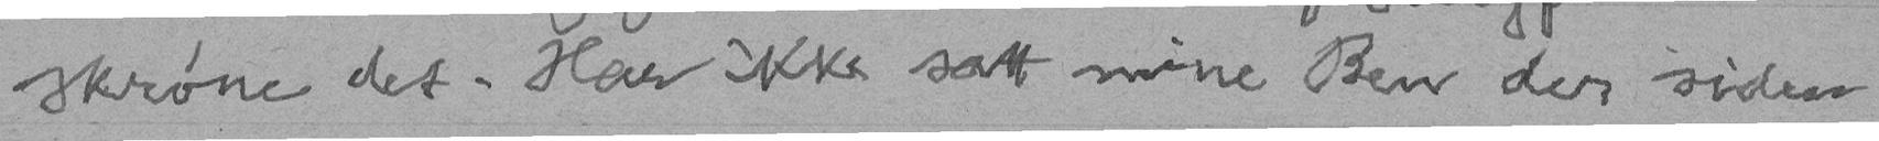skrøne det. Har ikke satt mine Ben der siden
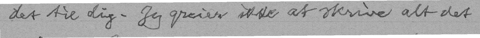det til dig. Jeg greier ikke at skrive alt det
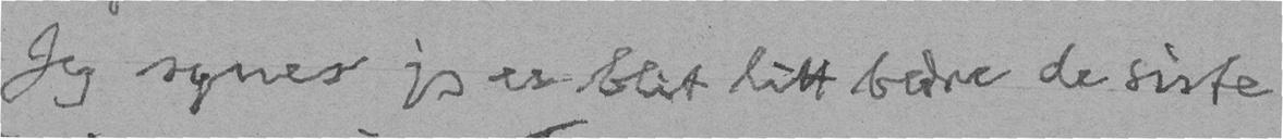Jeg synes jeg er blit litt bedre de siste
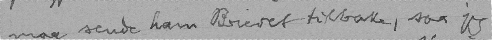maa sende ham Brevet tilbake, saa jeg
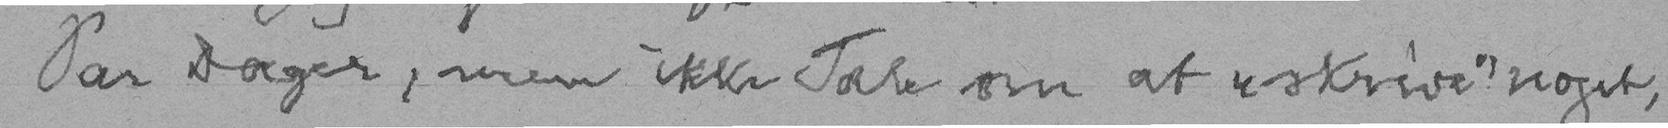Par Dager, men ikke Tale om at "skrive" noget,
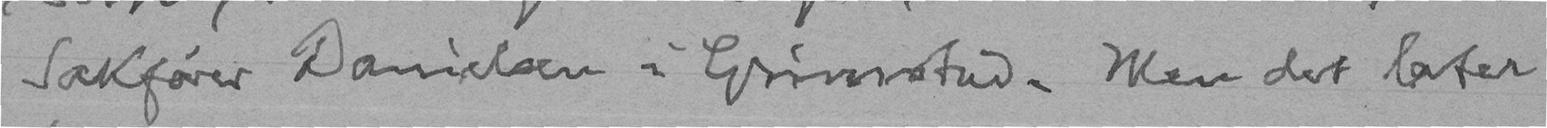Sakfører Danielsen i Grimstad. Men det later
